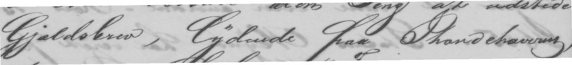Gjældsbrev,  lydende paa Phandehaveren,
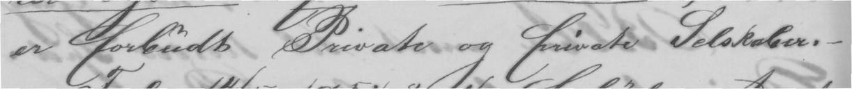er forbudt. Private og private Selskaber. -
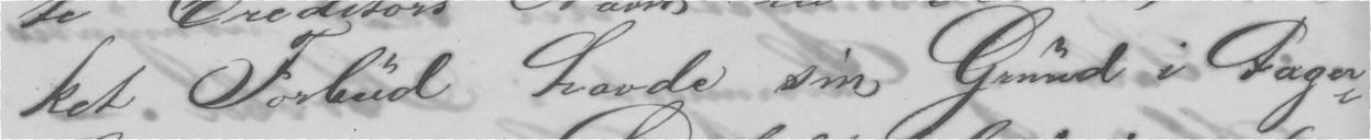ket Forbud havde sin Grund i tager-
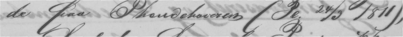de paa Phendelhaveren (Pe. 24/3 1811),
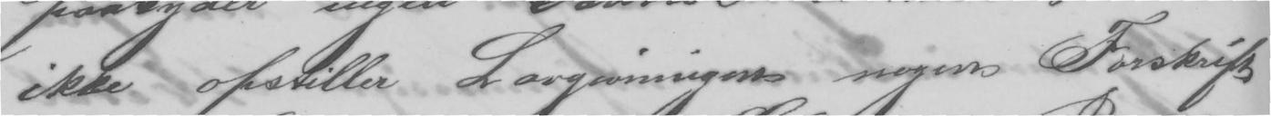ikke opstiller Lovgivningen nogen Forskrift
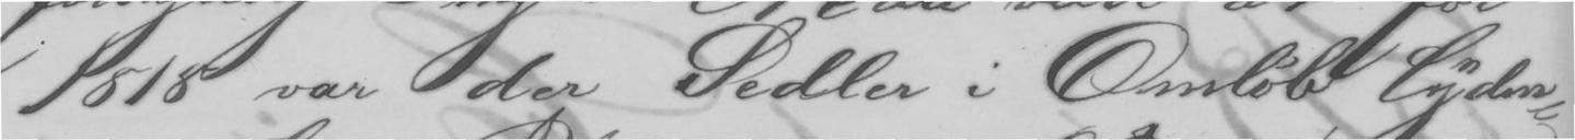1818 var der Sedler i Omløb lyden-
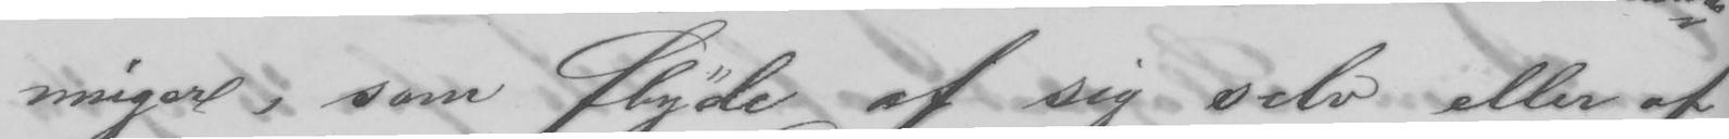mugerl, som flyde af sig selv eller af
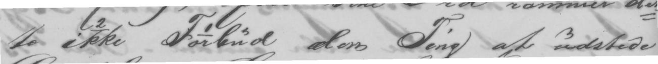te ikke Forbud den Ting at udstede
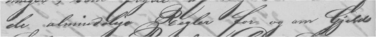de almindelige Regler for og om Gjelds
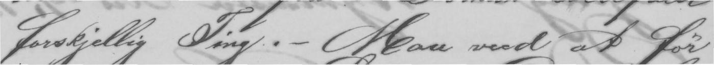Forskjellig Ting. - Man ved at før
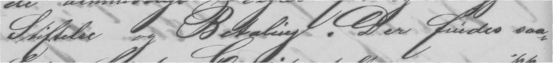Stiftelse og Betaling. Der findes saa-
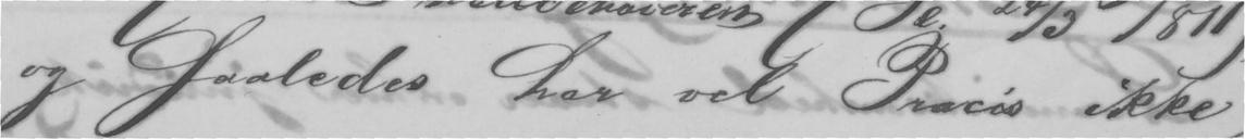og saaledes har vel praxis ikke
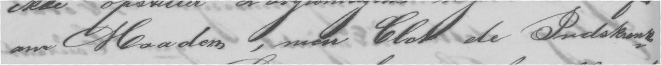om Maaden, men blot de Predskrank-
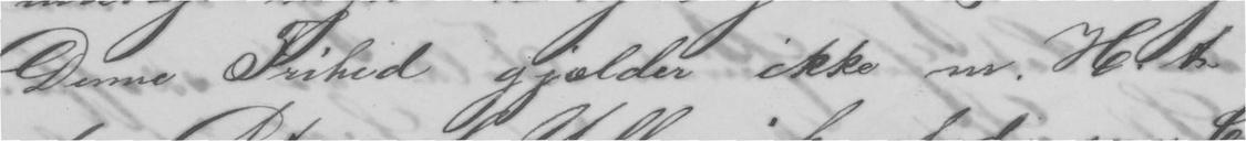Denne Frihed gjælder ikke m.H.t.
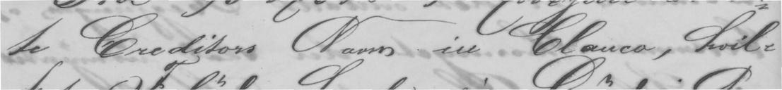te Criditors Navn in blanco, hvil-
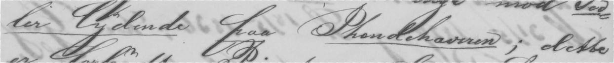ler lydende paa Phendehaveren; dette
